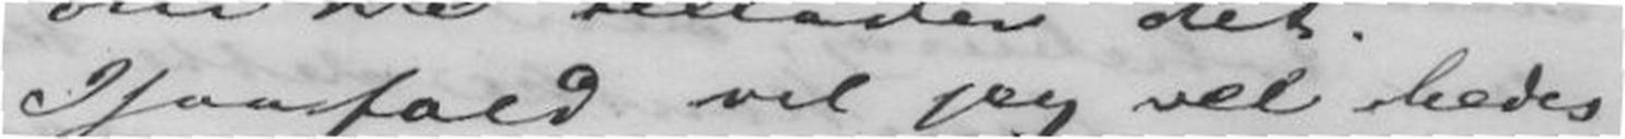Isaafald vil jeg vel bedes
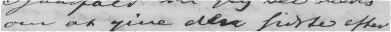om at give den sidste efter
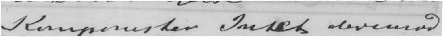Komponister Intet derimod
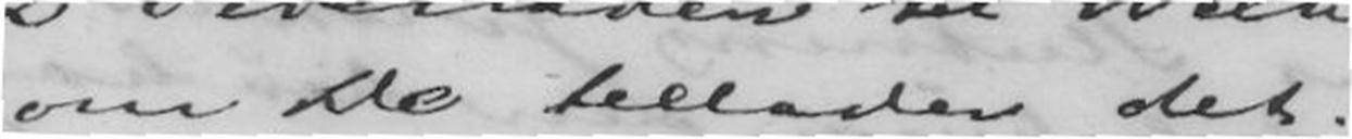om De tillader det.
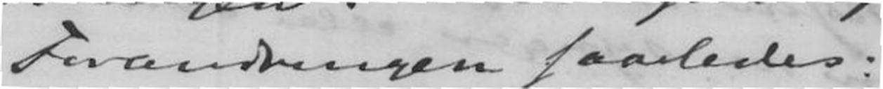Forandringen saaledes:
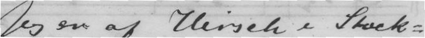Jeg er af Hirsch i Stock=
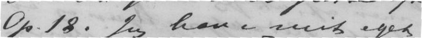Op. 18. Jeg har i mit eget
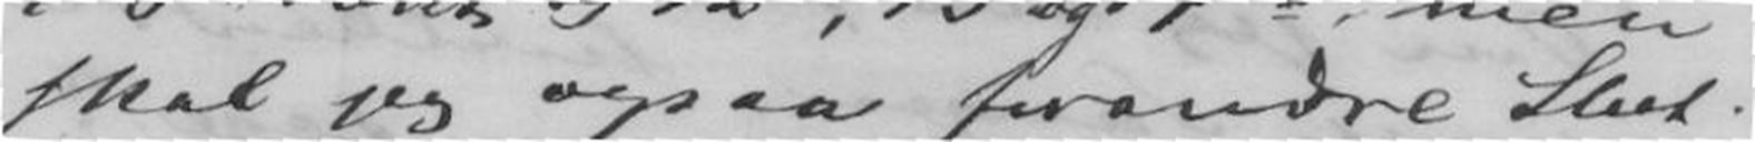skal jeg ogsaa forandre Slut
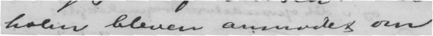holm bleven anmodet om
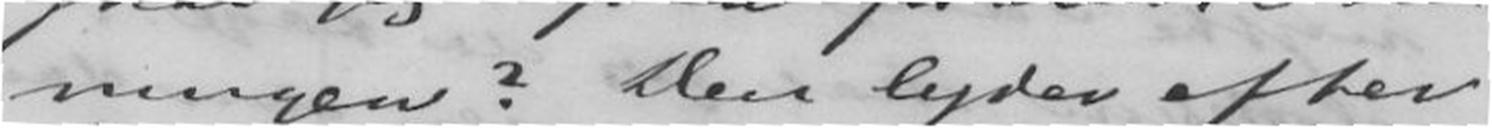ningen? Den lyder efter
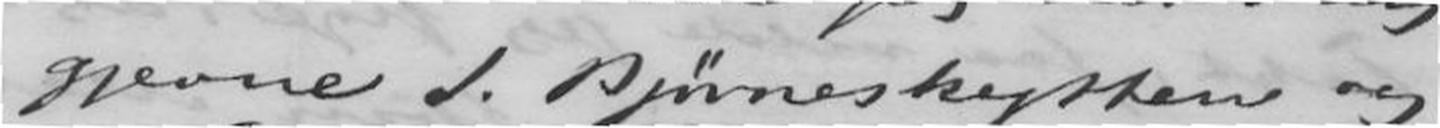gjerne 1. Bjørneskytten og
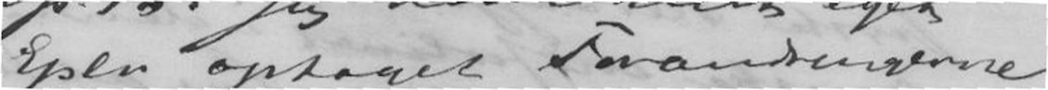Eplr. optaget Forandringerne
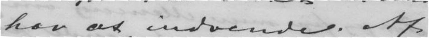har at indvende. Af
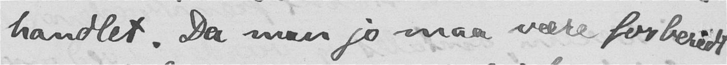handlet. Da man jo maa være forberedt
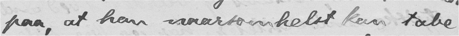paa, at han naarsomhelst kan tabe
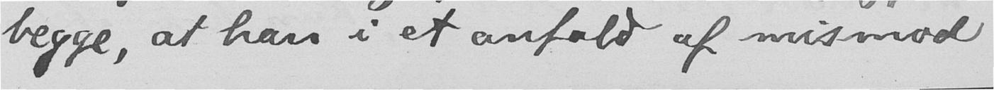begge, at han i et anfald af mismod
